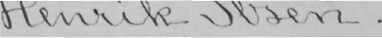Henrik Ibsen.
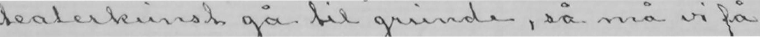teaterkunst gå til grunde, så må vi få
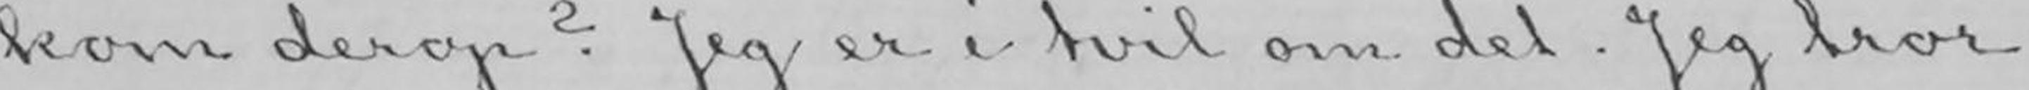kom derop? Jeg er i tvil om det. Jeg tror
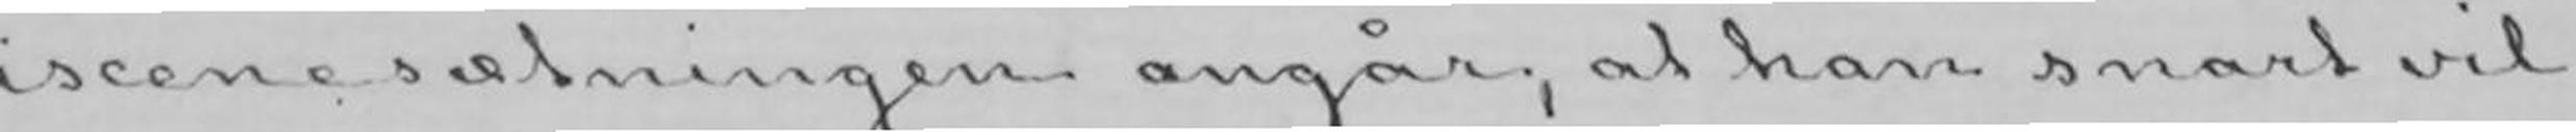iscenesætningen angår, at han snart vil
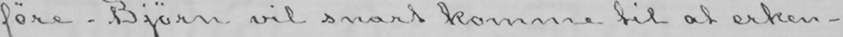føre. Bjørn vil snart komme til at erken-
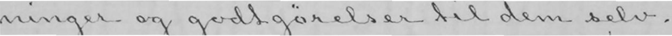ninger og godtgørelser til dem selv.
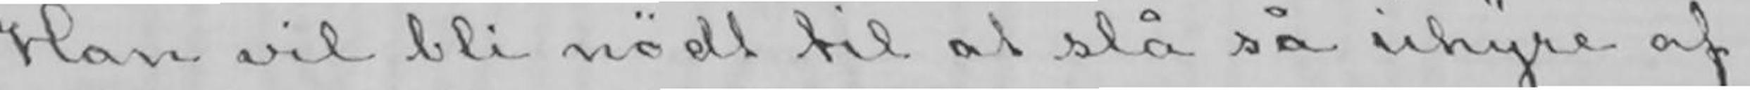Han vil bli nødt til at slå så uhyre af
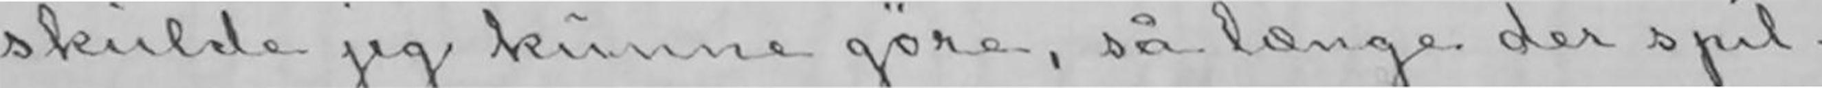skulde jeg kunne gøre, så længe der spil-
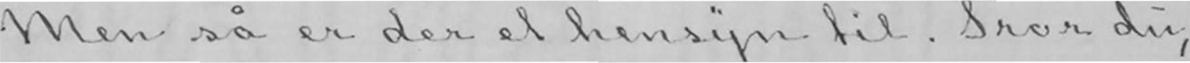Men så er der et hensyn til. Tror du,
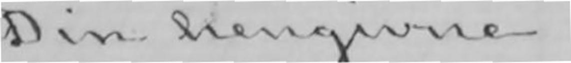Din hengivne
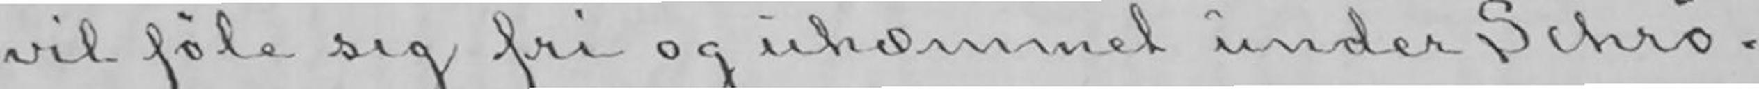vil føle sig fri og uhæmmet under Schrø-
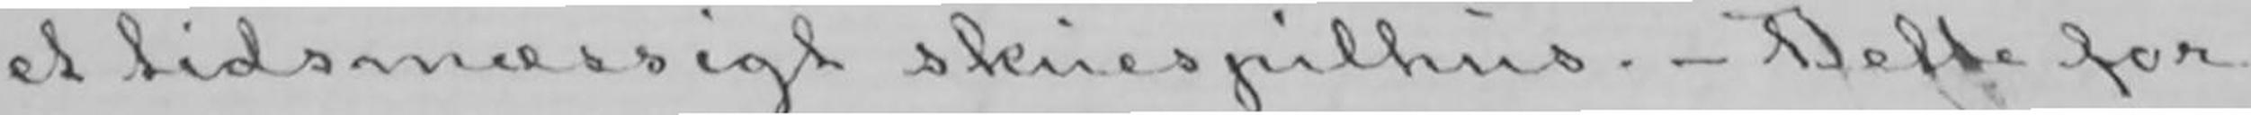et tidsmæssigt skuespilhus.- Dette for
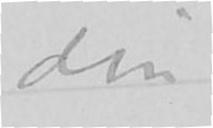din
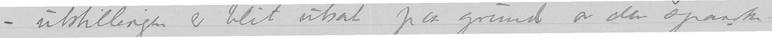utstillingen er blit utsat paa grund av den spanske.
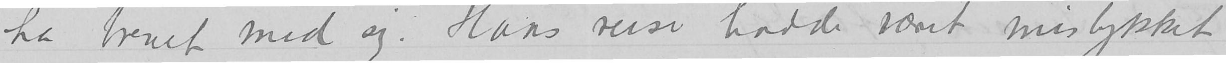ha brevet med sig. Hans reise hadde været mislykket –
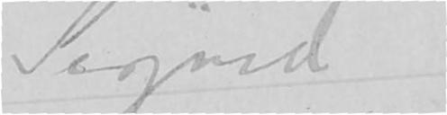Sigrid
